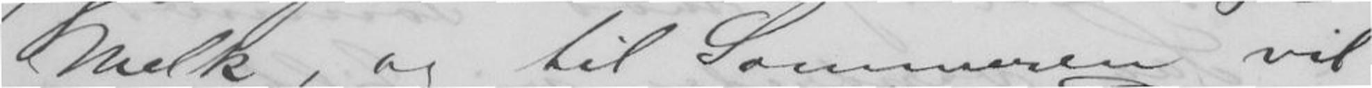Melk, og til Sommerem vil
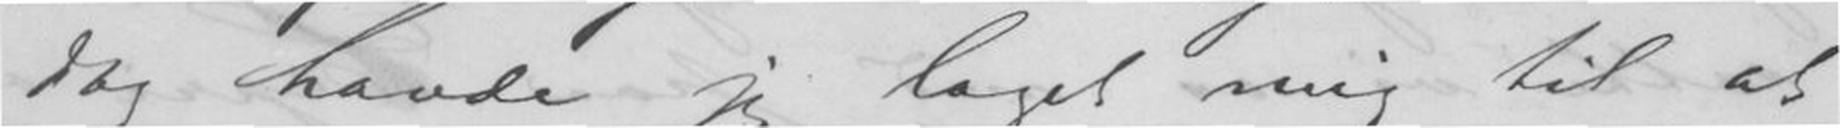dag havde jeg laget mig til at
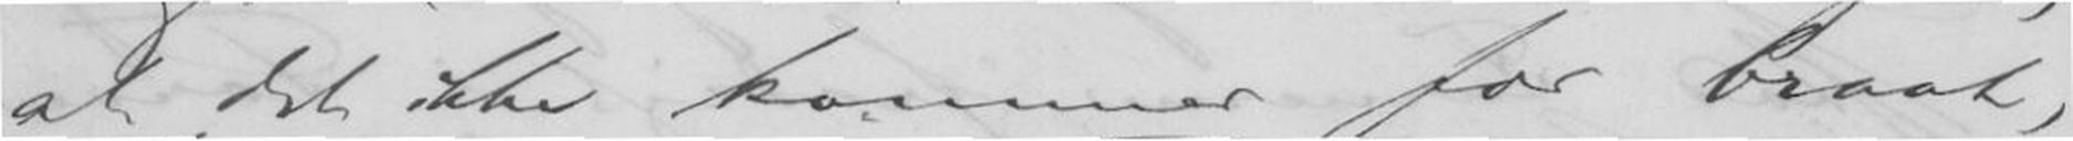at det ikke kommer for braat,
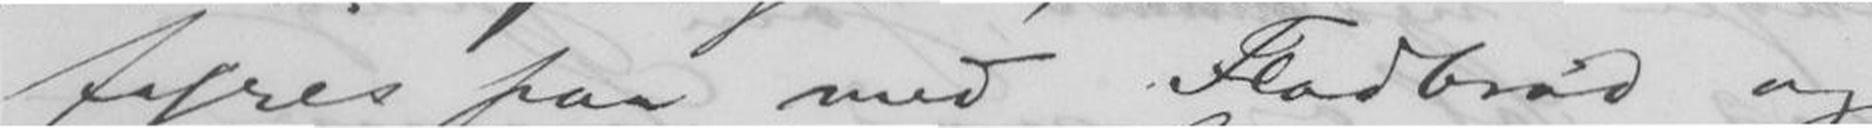fyres paa med Fladbrød og
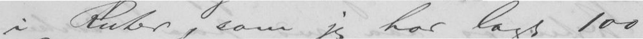i Ruter, som jeg har lagt 100
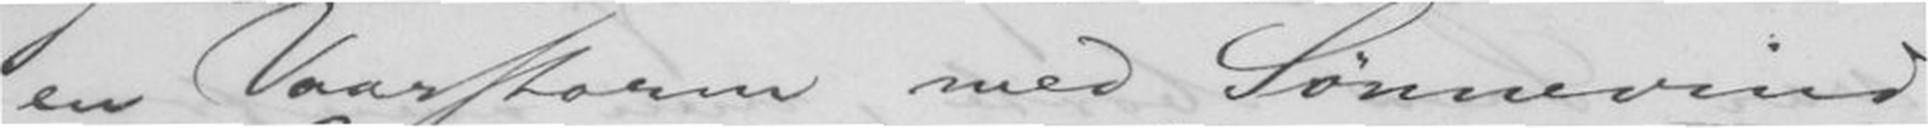en Vaarstorm med Sønnevind
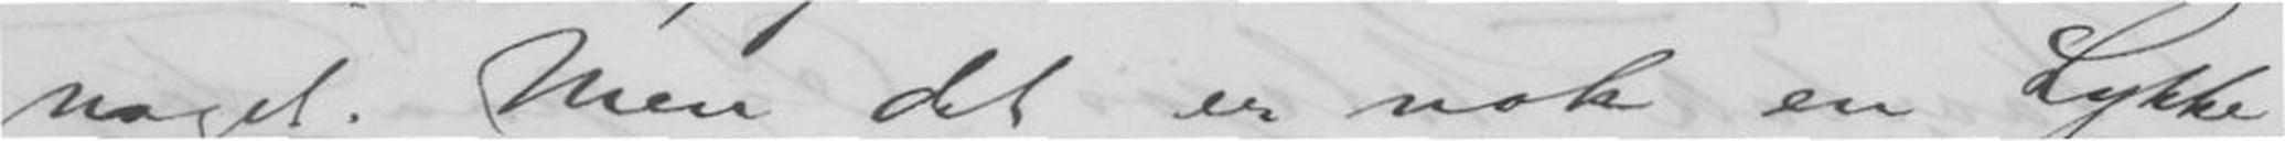noget. Men det er nok en Lykke,
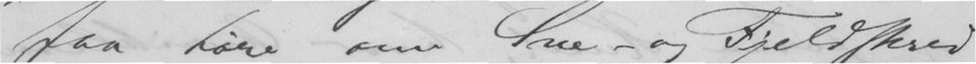faa høre om Sne- og Fjeldskred
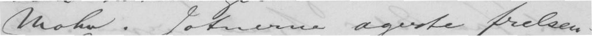Mohn. Jotnerne agerte frelsen-
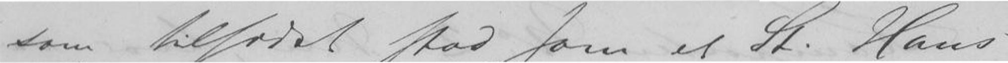som tilsidst stod som et St. Hans-
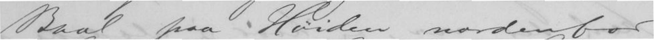Baal paa Høiden nordenfor
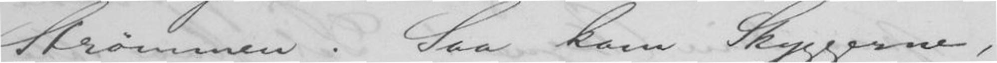Strømmen. Saa kom Skyggerne,
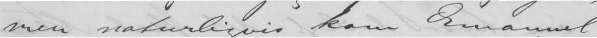men naturligvis kom Emanuel
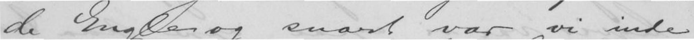de Engle og snart var vi inde
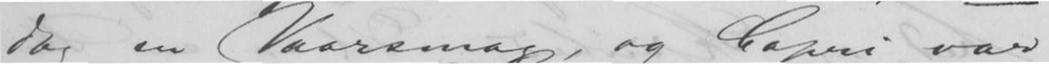dog en Vaarsmag, og Capri var
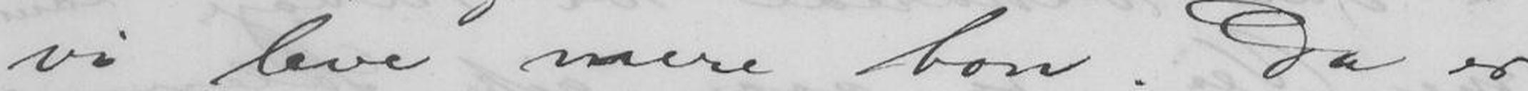vi leve mere bon. Da er
